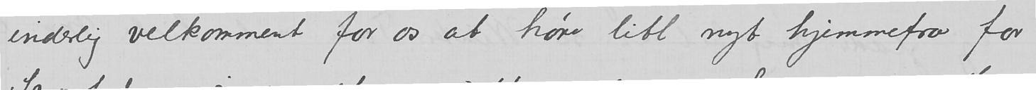inderlig velkomment for os at høre litt nyt hjemmefra for
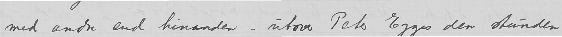med andre end hinanden – utover Peter Egges den stunden
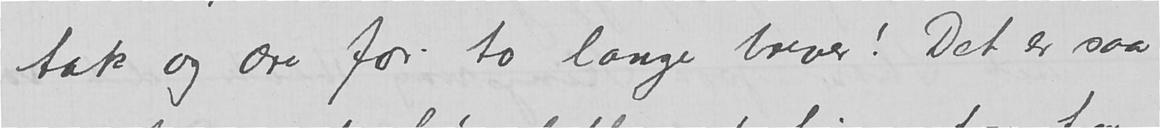tak og ære for to lange brever! Det er saa
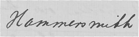Hammersmith
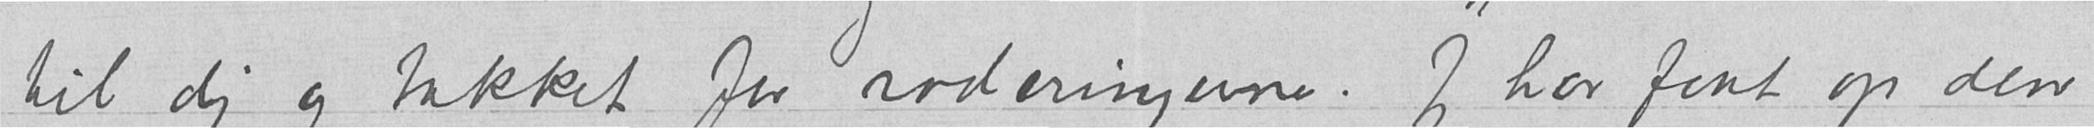til dig og takket for raderingerne. Jeg har faat op den
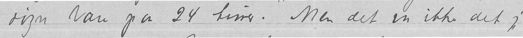døgn bare paa 24 timer. Men det var ikke det jeg
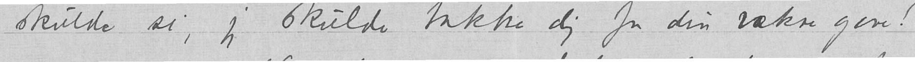skulde si, jeg skulde takke dig for din vakre gave!
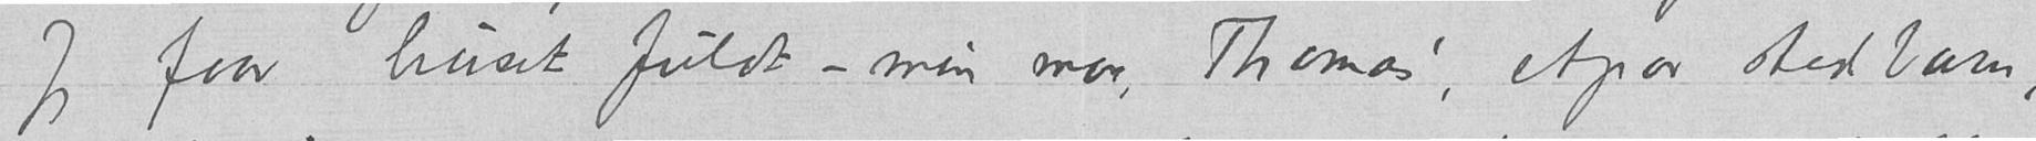Jeg faar huset fuldt – min mor, Thomas', etpar stedbarn,
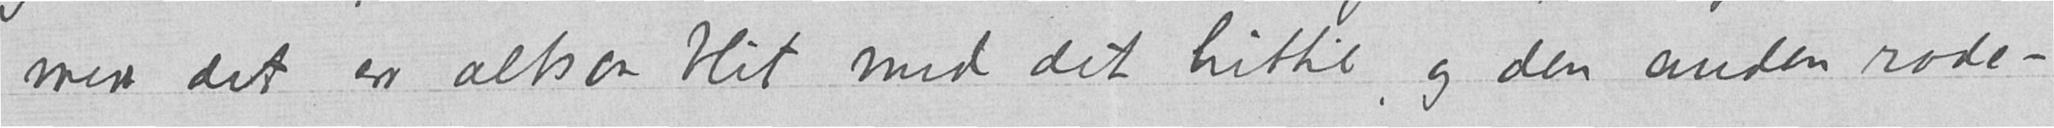men det er altsaa blit med det hittil, og den anden rade-
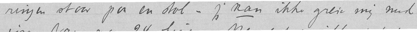ringen staar paa en stol – jeg kan ikke greie mig med
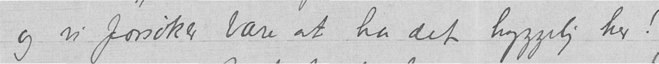og vi forsøker bare at ha det hyggelig her!
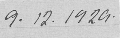9.12.1929
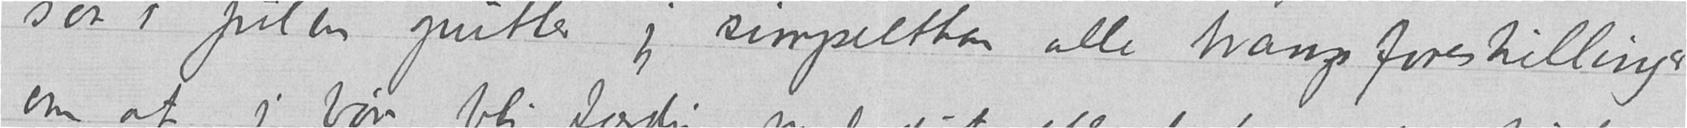saa i julen putter jeg simpelthen alle tvansforestillinger
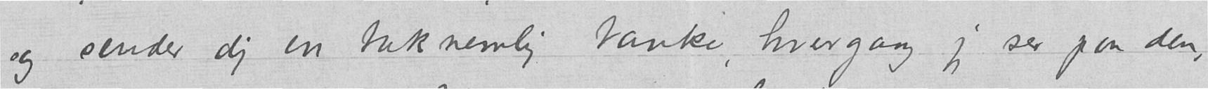og sender dig en taknemlig tanke, hvergang jeg ser paa den,
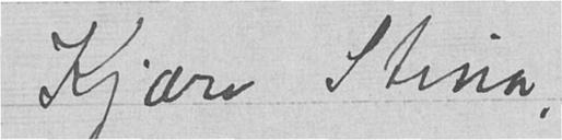Kjære Stina,
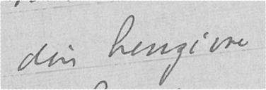din hengivne
